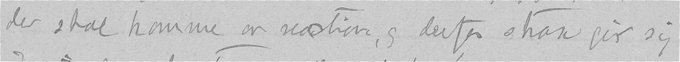der skal komme en reaction, og derfor strax gir sig
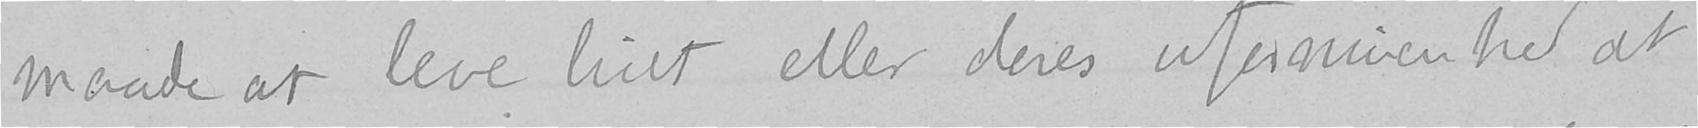maade at leve livet eller deres uformuenhed at
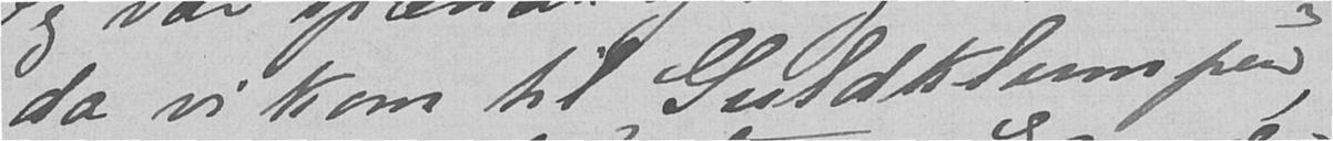da vi kom til "Guldklumpen",
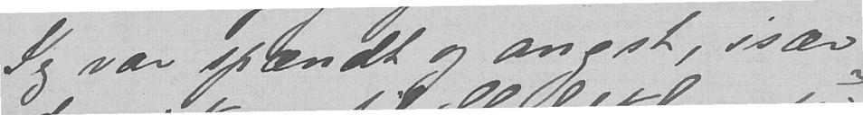Jeg var spændt og angst, især
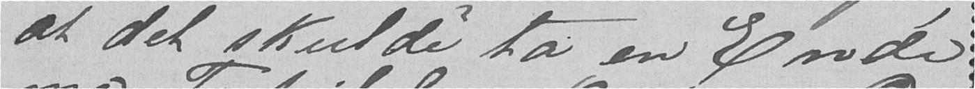at det skulde ta en Ende
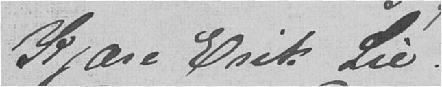Kjære Erik Lie.
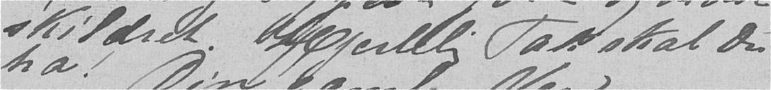skildret. Hjertelig Tak skal du
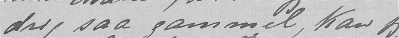drig saa gammel, kan jeg
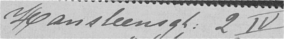Hansteensgt. 2 IV
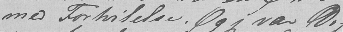med Fortvilelse. Og jeg var Dig
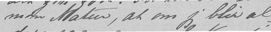min Natur, at om jeg blir al-
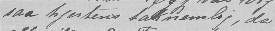saa hjertens taknemlig, da
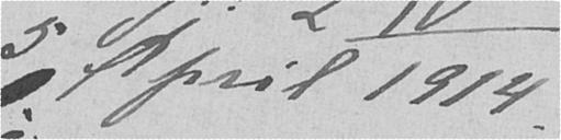5 April 1914.
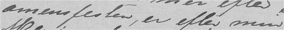amensfesten, er efter min
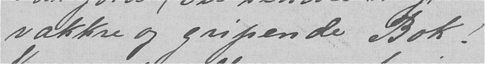vakkre og gripende Bok!
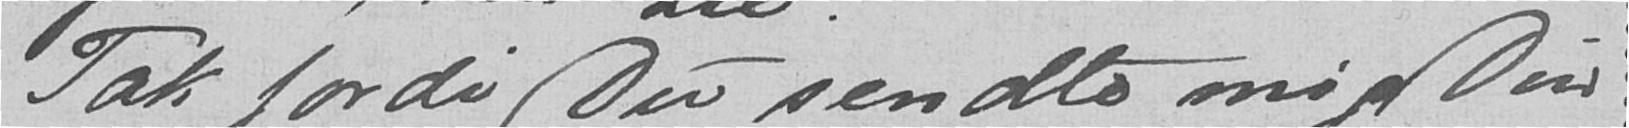Tak fordi Du sendte mig Din
